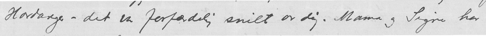Hardanger – det var forfærdelig snilt av dig. Mama og Signe har
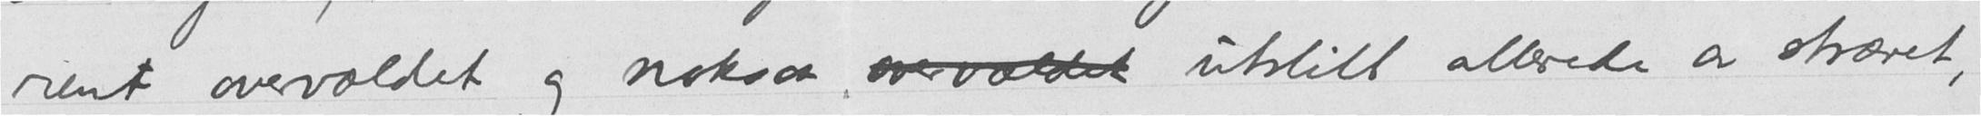rent overvældet og noksaa overvældet utslitt allerede av strævet,
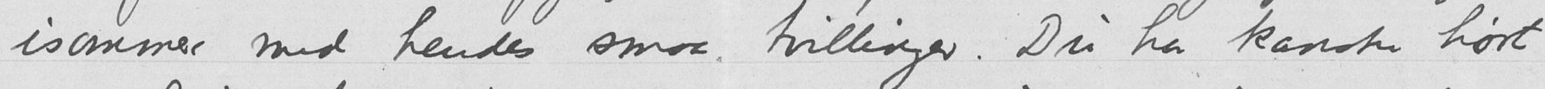isommer med hendes smaa tvillinger. Du har kanske hørt
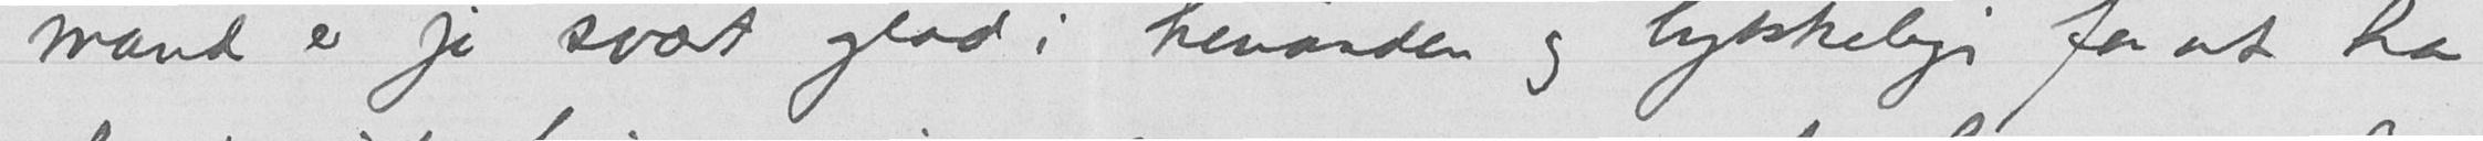mand er jo svært glad i hinanden og lykkelige for at ha
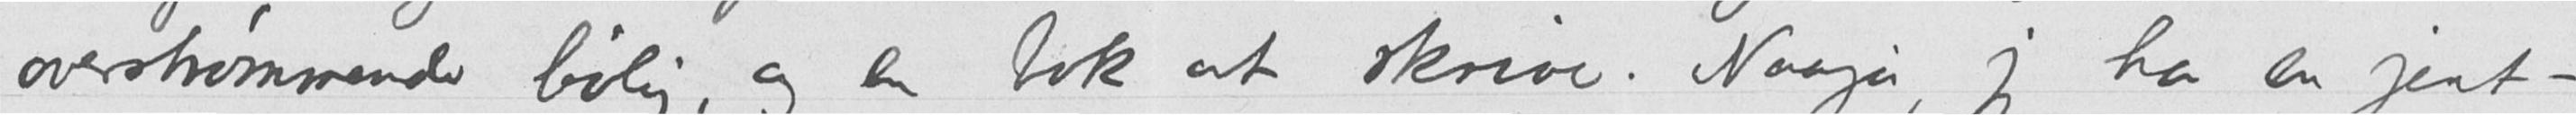overstrømmende livlig, og en bok at skrive. Naaja, jeg har en jent-
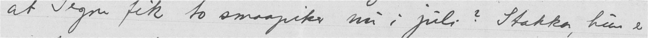at Signe fik to smaapiker nu i juli? Stakkar, hun er
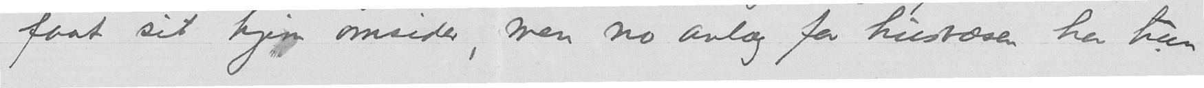faat sit hjem omsider, men no anlæg for husvæsen har hun
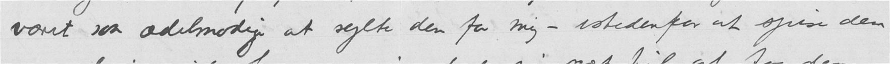været saa ædelmodige at sylte dem for mig – istedenfor at spise dem
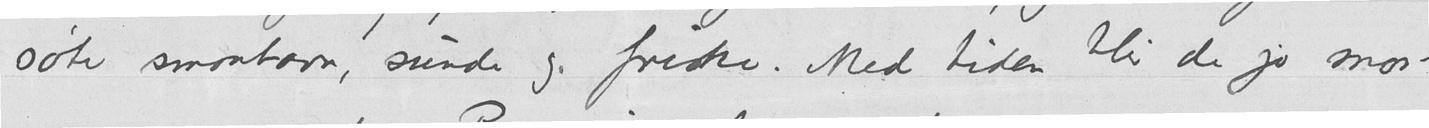søte smaabarn, sunde og friske. Med tiden blir de jo mor-
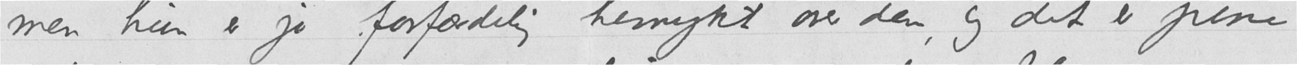men hun er jo forfærdelig henrykt over dem, og det er pene
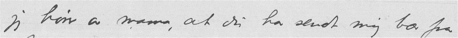jeg hører av mama, at du har sendt mig bær fra
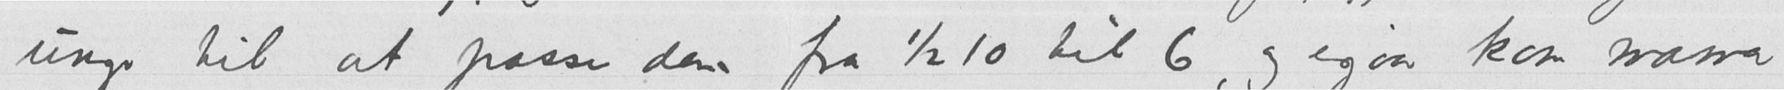unge til at passe dem fra ½ 10 til 6, og igaar kom mama
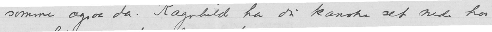somme ogsaa da. Ragnhild har du kanske set nede hos
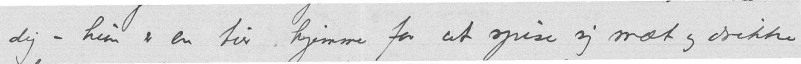dig – hun er en tur hjemme for at spise sig mæt og drikke
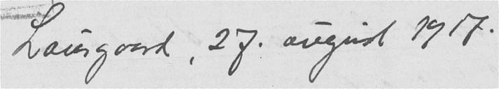Laurgaard, 27. august 1917.
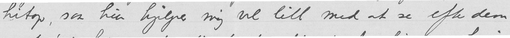hitop, saa hun hjelper mig vel litt med at se efter dem
